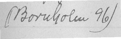(Bornholm 96)
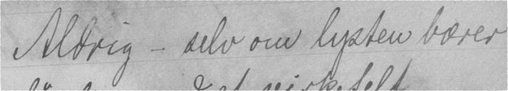Aldrig - selv om lysten bærer
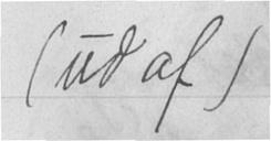(ud af)
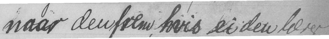naar den frem, hvis ei den lærer
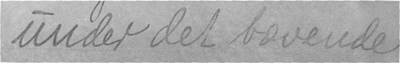under det bævende
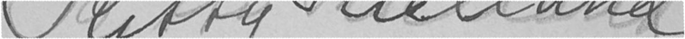Kitty Kielland
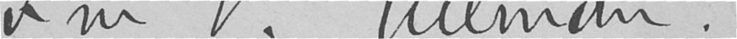Fru V. Ullman.
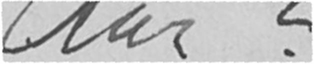Rue ?
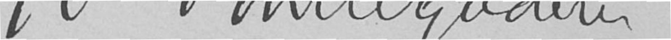10 Tomtegaden
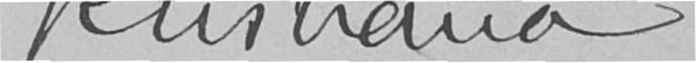Kristiania
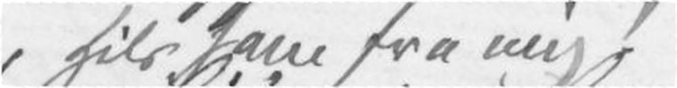Hils ham fra mig!
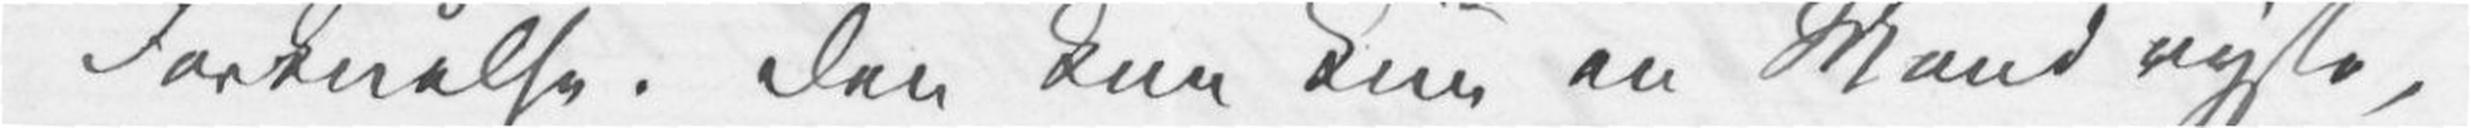Forkuelse. Den kan kun en Mand nyte,
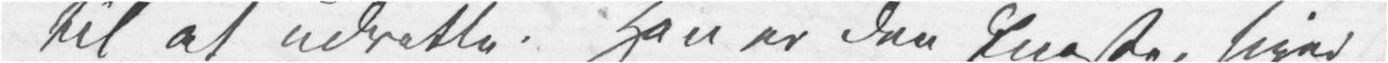til at udrette! Han er den Eneste, siger
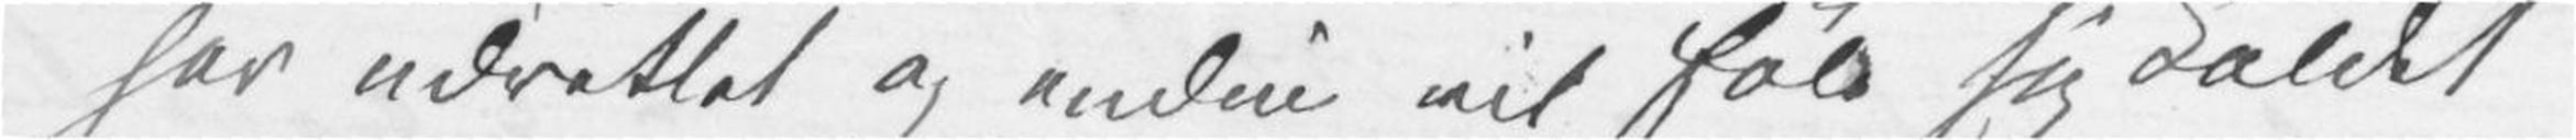har udrettet og endnu vil føle sig kaldet
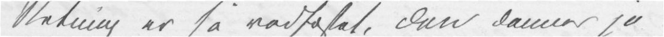Retning er så rodfæstet. Den danner jo
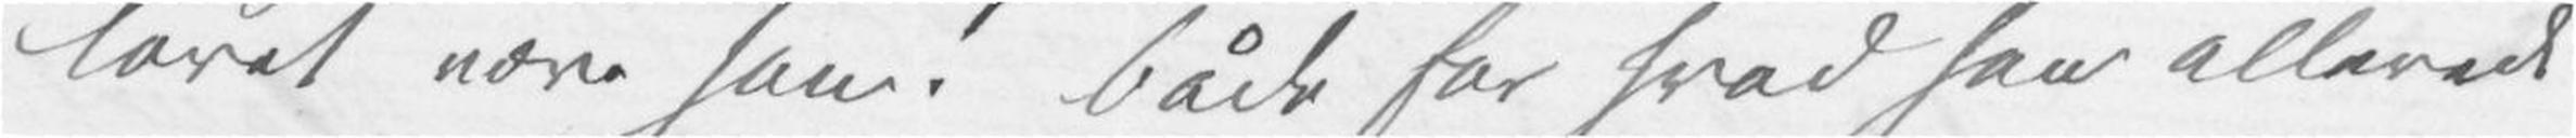lovet være ham! Både for hvad han allerede
